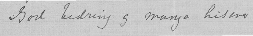God bedring og mange hilsener
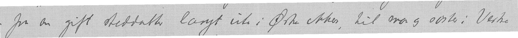- fra en gift steddatter langt ute i Østre Aker, til mor og søster i Vestre
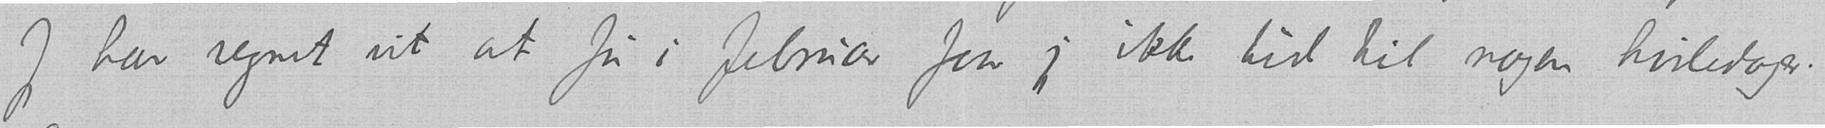Jeg har regnet ut at før i februar faar jeg ikke tid til nogen hviledager.
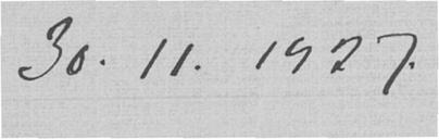30.11.1927.
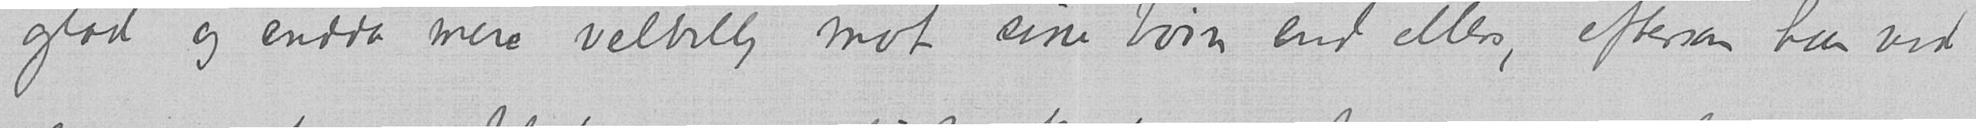glad og endda mere velvillig mot sine børn end ellers, eftersom har ved
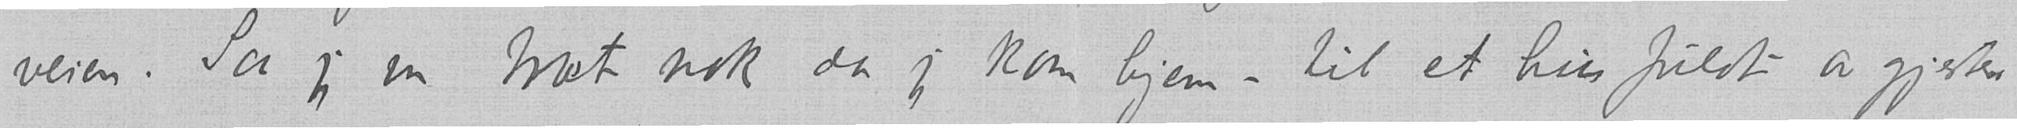veien. Saa jeg var træt nok da jeg kom hjem – til et hus fuldt av gjester.
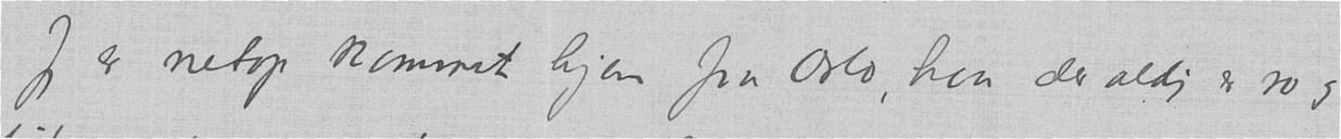Jeg er netop kommet hjem fra Oslo, hvor der aldrig er ro og
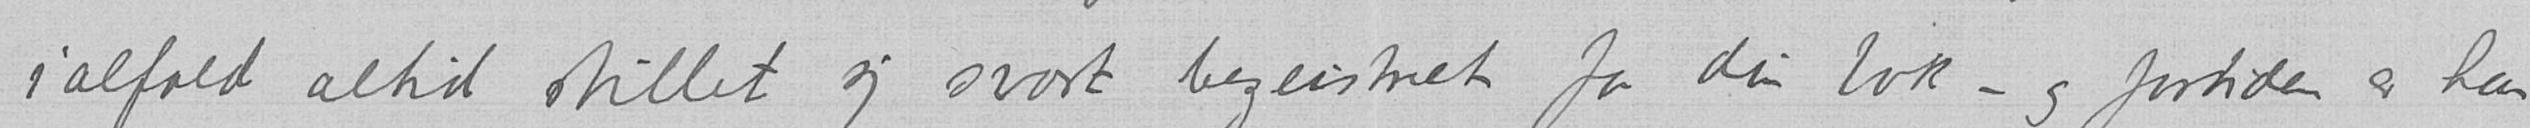ialfald altid stillet sig svært begeistret for din bok – og fortiden er han
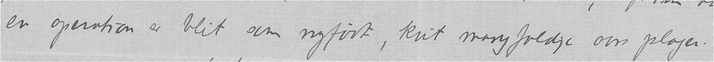en operation er blit som nyfødt, kvit mangfoldige aars plager.
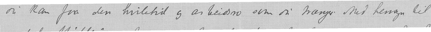du kan faa den hviletid og arbeidsro som du trænger. Med hensyn til
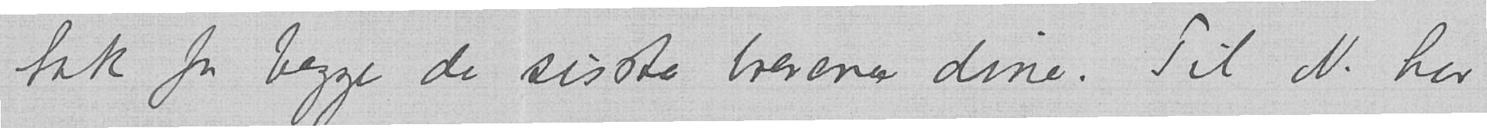tak for begge de sisste brevene dine. Til N. har
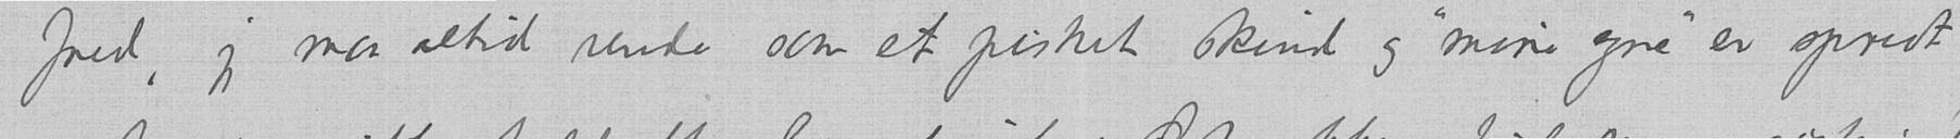fred, jeg maa altid rende som et pisket skind og "mine egne" er spredt
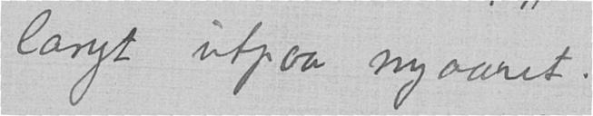langt utpaa nyaaret.
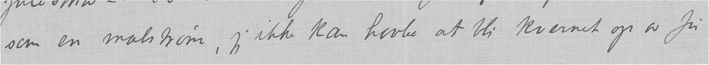som en malstrøm, jeg ikke kan haabe at bli kvernet op av før
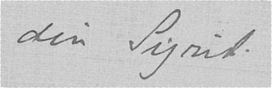din Sigrid.
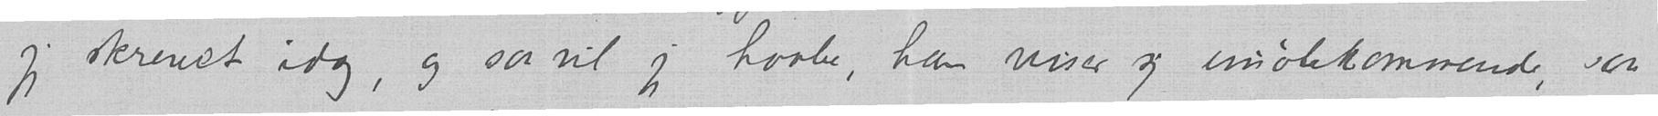jeg skrevet idag, og saa vil jeg haabe, han viser sig imøtekommende, saa
